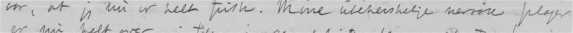var, at jeg nu er helt frisk. Mine ubeherskelige nervøse plager
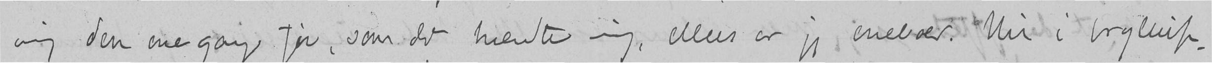mig den ene gang før, som det hendte mig, ellers er jeg eneboer. Nu i bryllup-
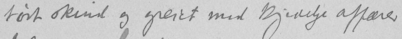tørt skind og greiet med kjedelige affærer
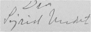Sigrid Undset
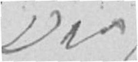Din
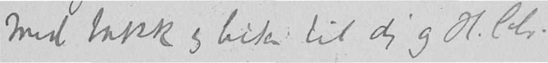Med takk og hilsen til dig og H. Chr.
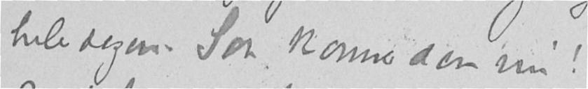hele dagen. Saa kommer den nu!
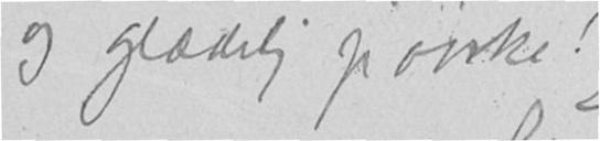og glædelig paaske!
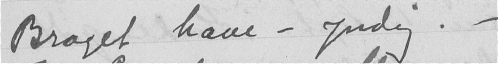Broget have - yndig. -
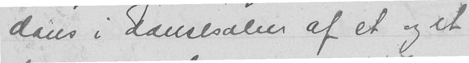dans i dansesalen af et og et
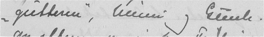"gutterne", Minni og Gunh.
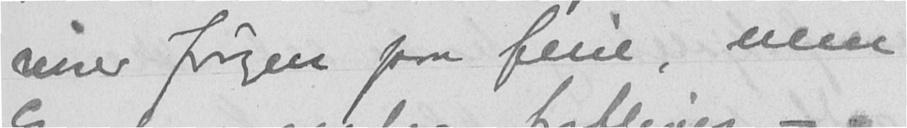reiser Jørgen paa ferie, men
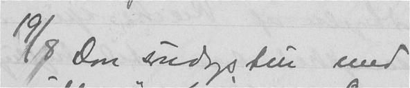19/8 Don søndagstur med
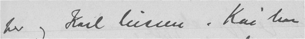her og Karl Nissen. Kai har
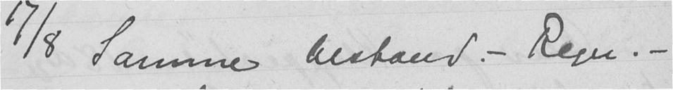17/8 Samme bestand. - Regn. -
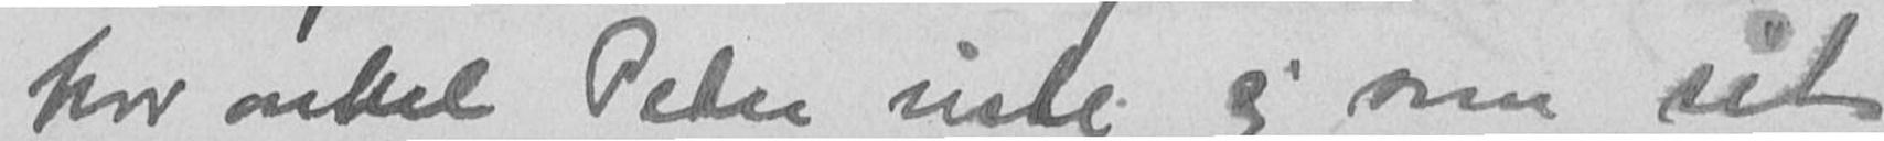hvor onkel Peder viste sig som sit
